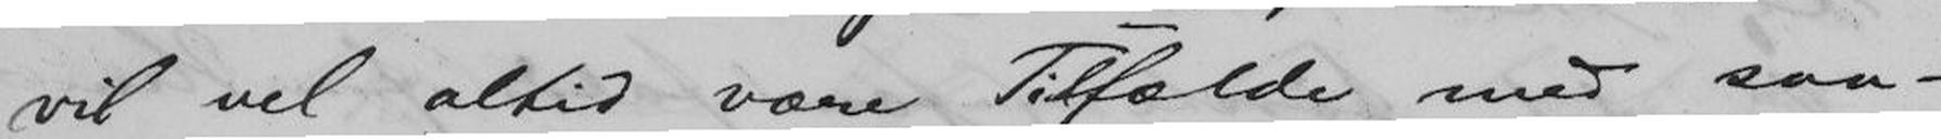vil vel altid være Tilfælde med saa-
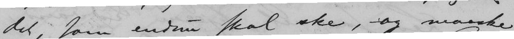det, som endnu skal ske, og maaske
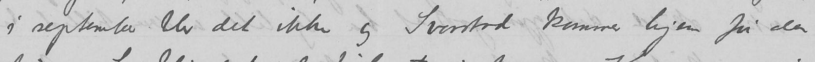i september blir det ikke og Svarstad kommer hjem før den
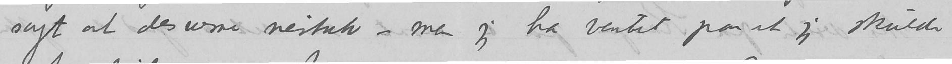sagt at deværre neitak – men jeg har ventet paa at jeg skulde
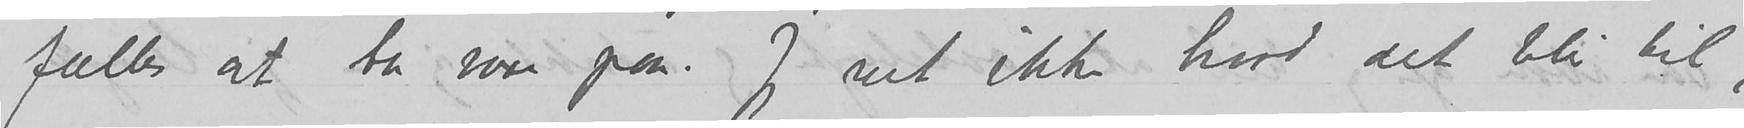fælles at ta være paa. Jeg vet ikke hvad det blir til,
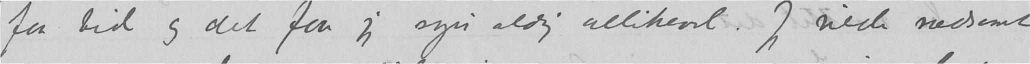faa tid og det faar jeg sgu aldrig allikevel. Jeg vilde rædsomt
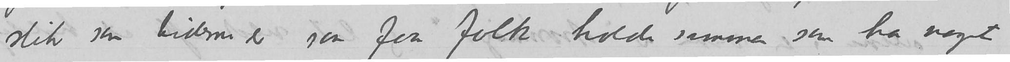slik som tiderne er saa faa folk holde sammen som har noget
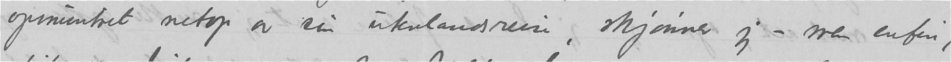opmuntret netop av sin utenlandsreise, skjønner jeg – men enfin,
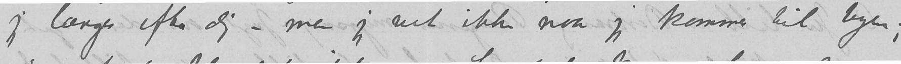jeg længes efter dig – men jeg vet ikke naar jeg kommer til byen;
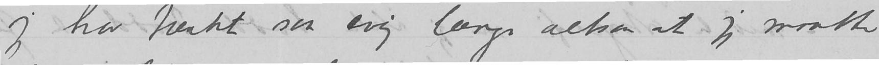jeg har tænkt saa evig længe altsaa at jeg maatte
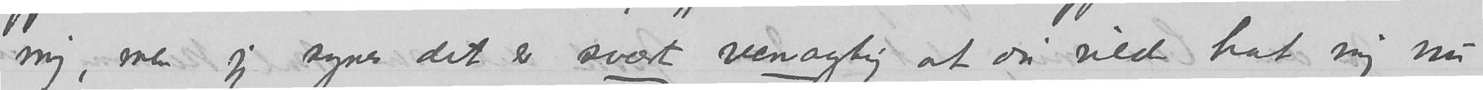mig, men jeg synes det er svært venagtig at du vilde hat mig nu
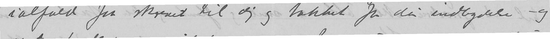ialfald faa skrevet til dig og takket for din indbydelse – og
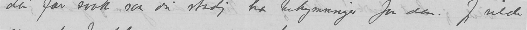du far svak saa du stadig har bekymringer for dem. Jeg vilde
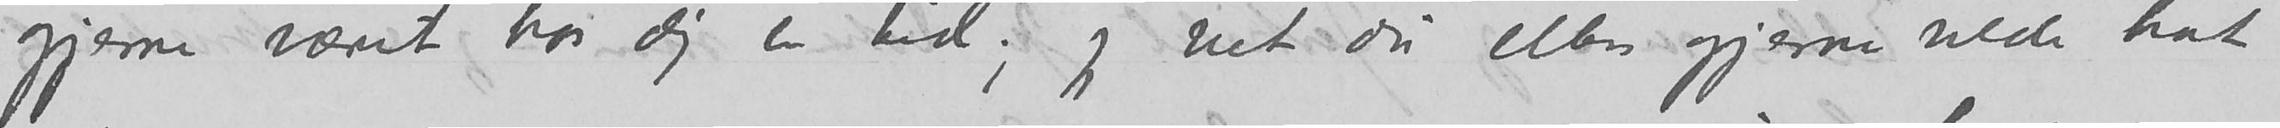gjerne været har dig en tid; jeg vet du ellers gjerne vilde hat
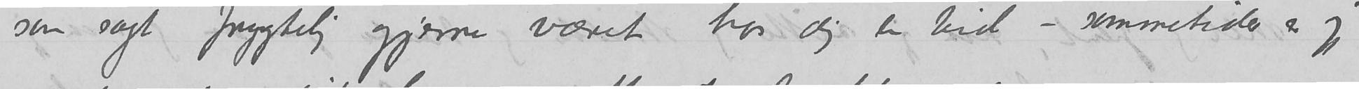som sagt frygtelig gjerne været har dig en tid – sommetider er jeg
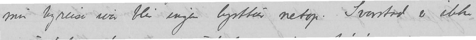min byreise svar blir ingen lysttur netop. Svarstad er ikke
